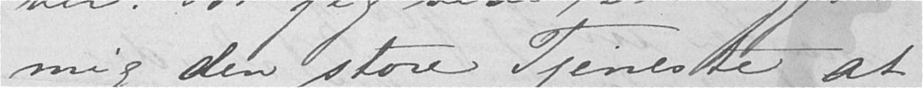mig den store Tjeneste at
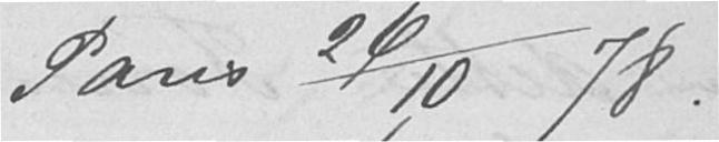Paris 26/10 78.
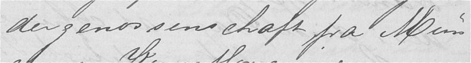dergenossenschaft fra Mün-
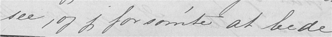see, og jeg forsømte at bede
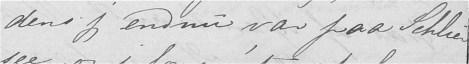dens jeg endnu var paa Schlier-
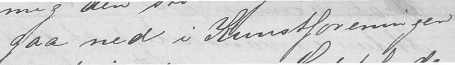gaa ned i Kunstforeningen
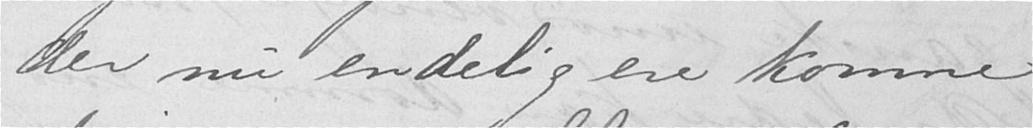der nu endelig ere komne
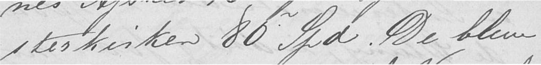sterkirken 80 Spd. De bleve
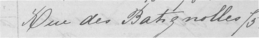Rue des Batignolles 75.
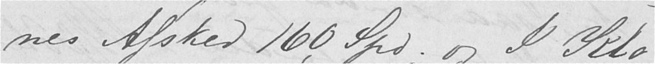nes Afsked 160 Spd. og I Klo-
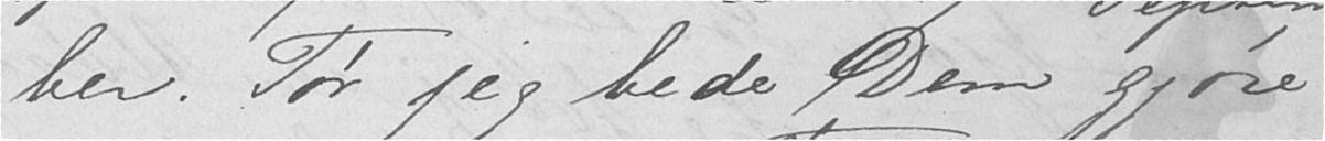ber. Tør jeg bede Dem gjøre
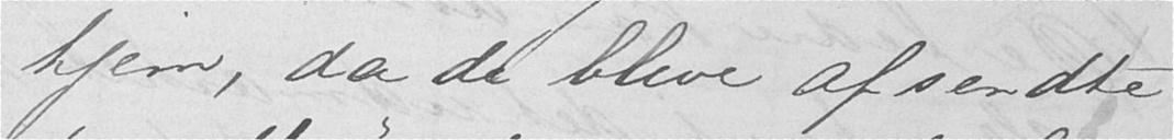hjem, da de bleve afsendte
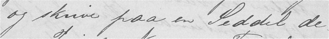og skrive paa en Seddel de-
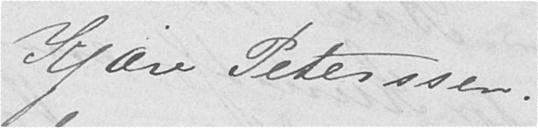Kjære Peterssen!
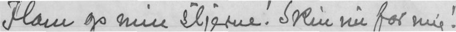Flom op min stjerne! Skin nu for mig!
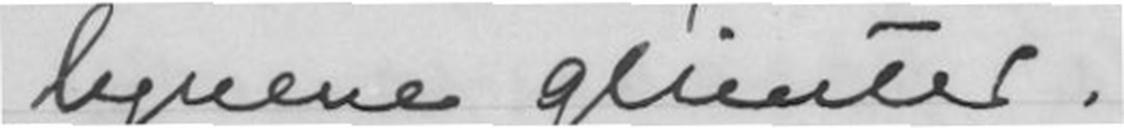lynene glimter.
